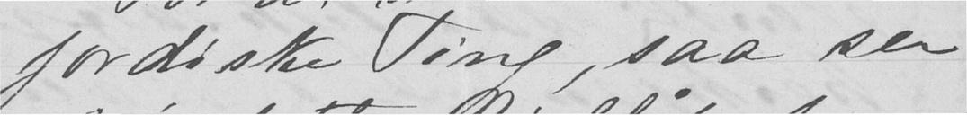jordiske Ting, saa ser
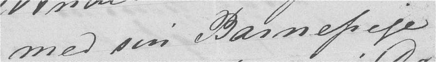med sin Barnepige
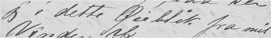jeg i dette Øieblik fra mit
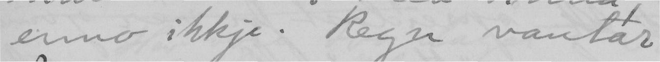enno ikkje. Regn vantar –
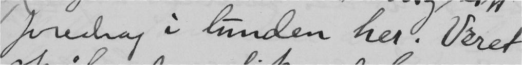foredrag i lunden her. Været
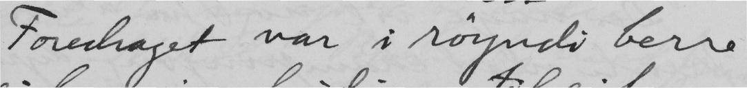Foredraget var i röyndi berre
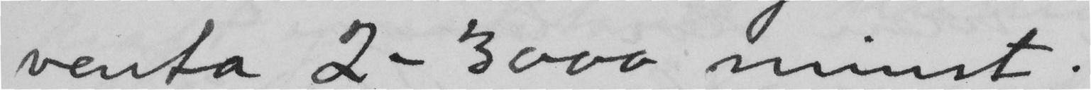venta 2-3000 minst.
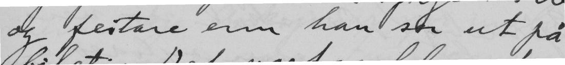og feitare enn han ser ut på
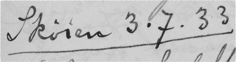Sköien 3.7.33
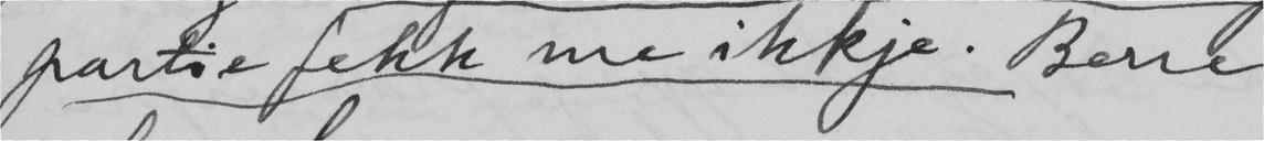partie fekk me ikkje. Berre
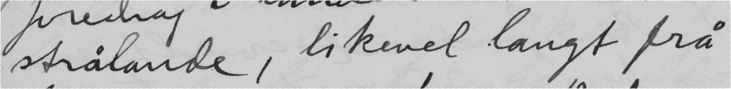strålande, likevel langt frå
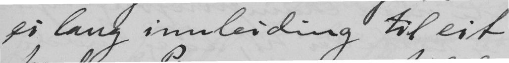ei lang innleiding til eit
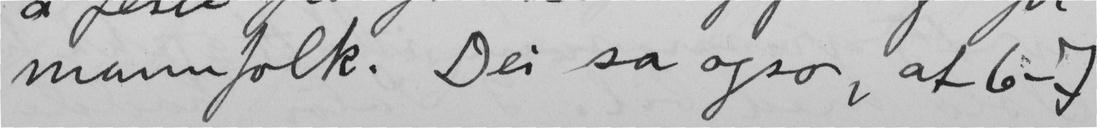mannfolk. Dei sa ogso, at 6-7
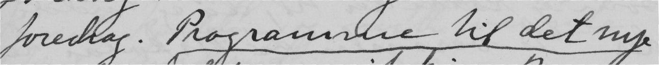foredrag. Programme til det nye
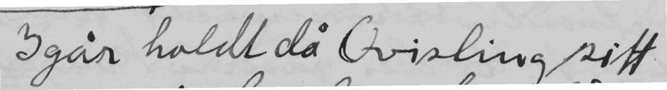Igår holdt då Qvisling sitt
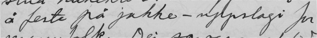å feste på jakke-uppslagi for
